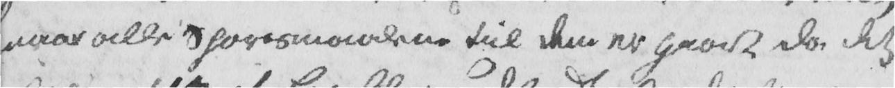naar alle Spørsmaalene til dem er giort da det
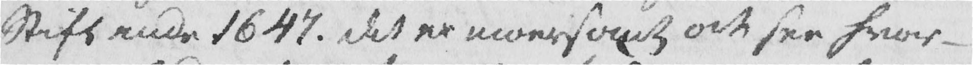Stift inde 1647. Det er moersomt at see hvor-
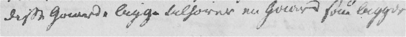disse Gaarde ligge tilhører en Gaard som ligger
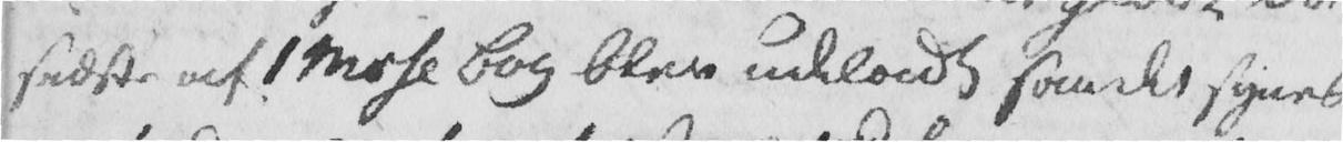sidste af 1 Messe Bog blev udeladt som det synes
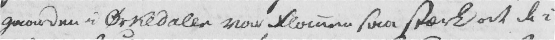gaarden i Ørkedalen var Flomen saa Stærk at de i
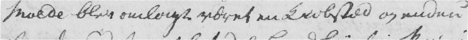Molde blev anlagt været en Kiøbstæd og endnu
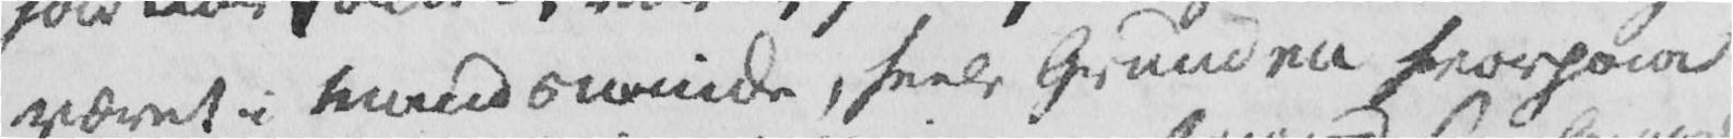været i Mandsminde, heele Grunden hvorpaa
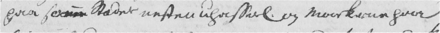paa some Stæder nesten upasserl. og Markerne paa
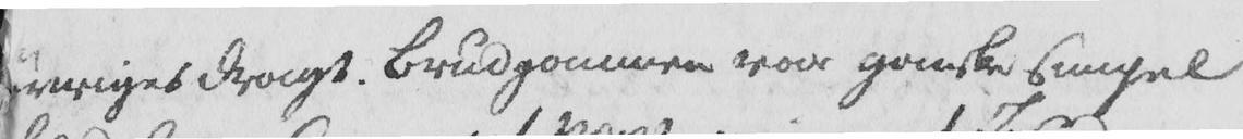øvriges Dragt. Brudgommen var ganske Simpel
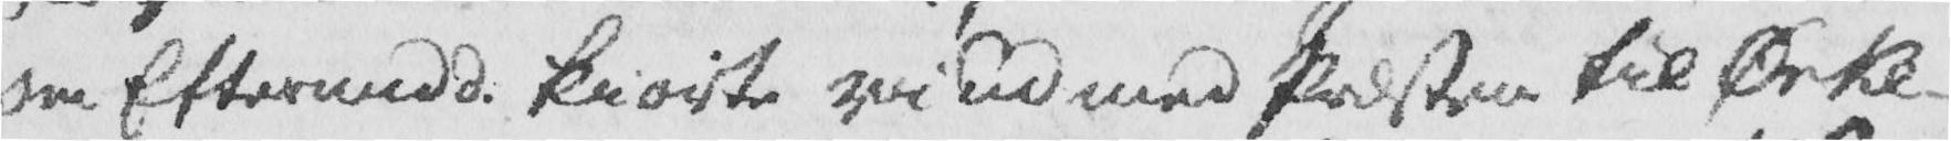Om eftermidd. kiørte vi ud med Præsten til Ørke-
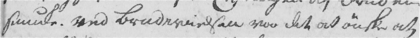smucke. Ved Brudevielsen var det at ønske at
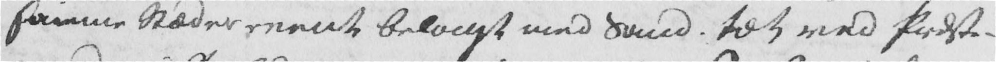samme Stæder reent belagt med Sand. tæt ved Præste-
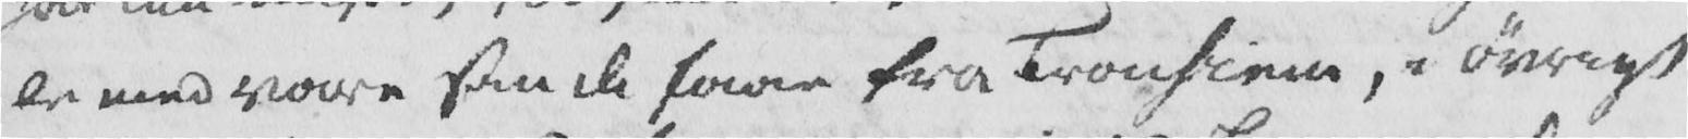le med Varer som de faae fra Tronhiem, i øvrigt
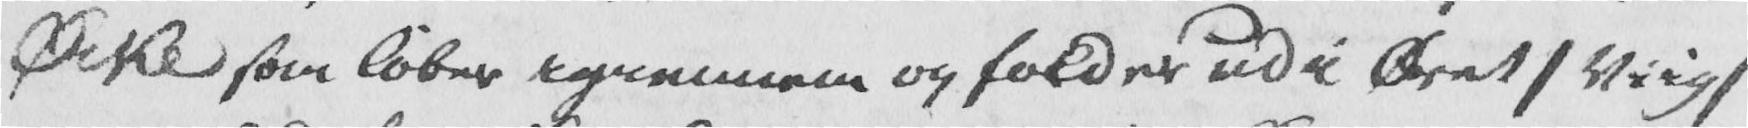Ørke som løber igiennem og falder udi Øret / Viig /
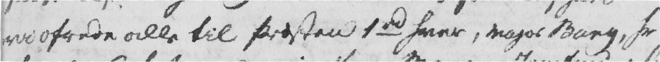vi ofrede alle til Præsten 1 rd hver, Major Bang, Hr
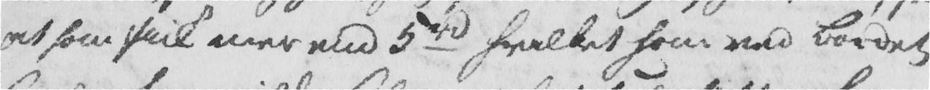at han fick mer end 5 rd hvilket han ved Bordet
